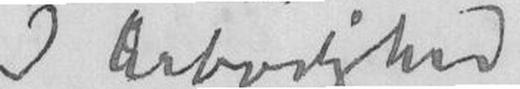I Ærbødighed
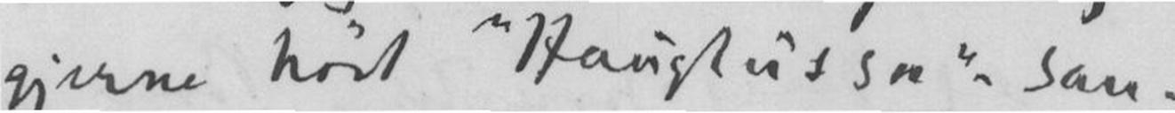gjerne hørt "Haugtussa" - San-
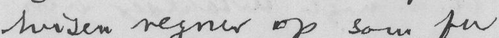Avisen regner op som for
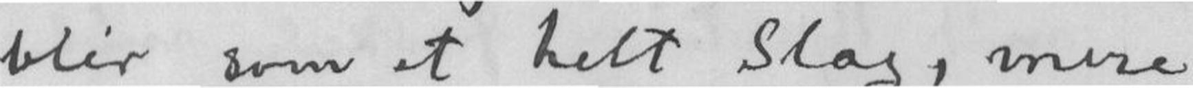blir som et helt Slag, mere
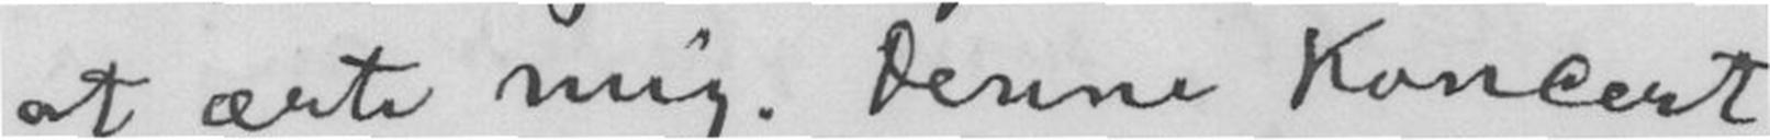at ærte mig. Denne Koncert
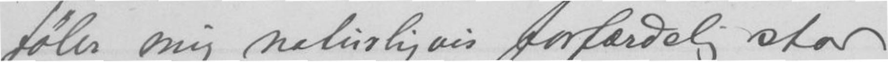føler mig naturligvis forfærdelig stor
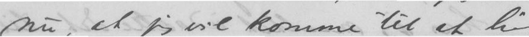nu, at jeg vil komme til at lige
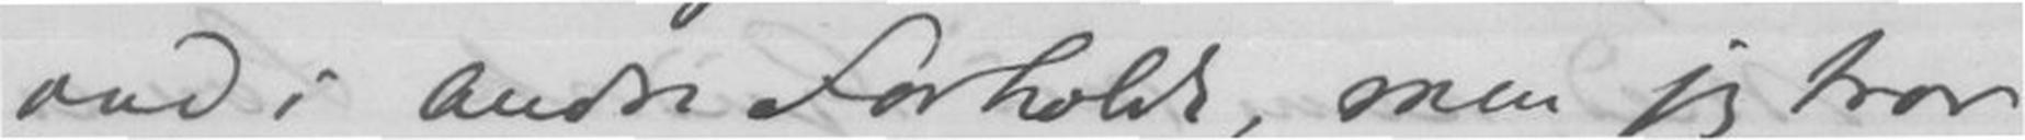ind i Andet Forholdet, men jeg tror
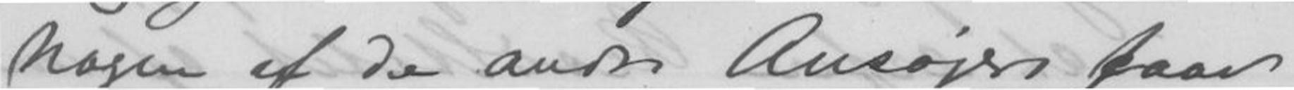Nogen af de andre Ansøgere faar
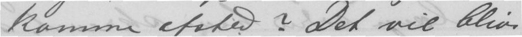komme afsted? Det vil blive
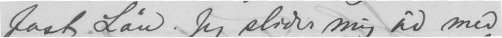fast Løn. Jeg slider mig ud med
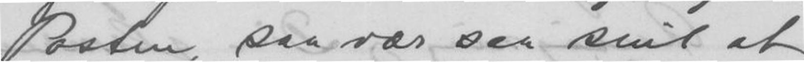Posten, saa vær saa snil at
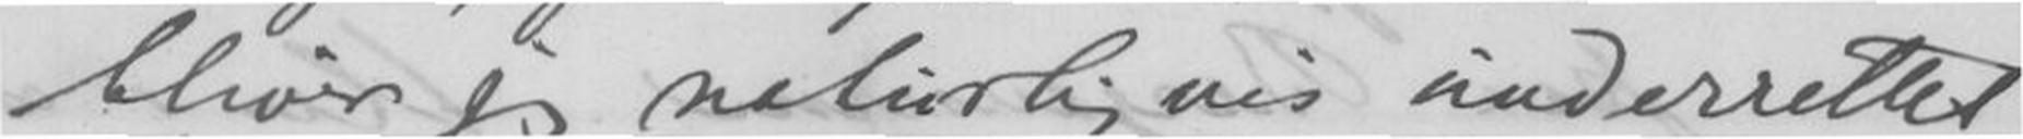bliver jeg naturligvis underrettet
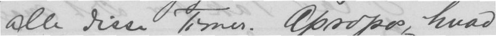alle disse Timer. Apropos, hvad
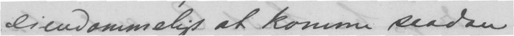eiendommeligt at komme saadan
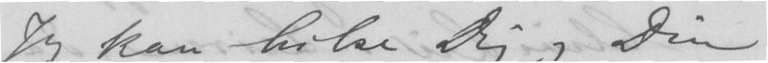Jeg kan hilse Dig og Din
